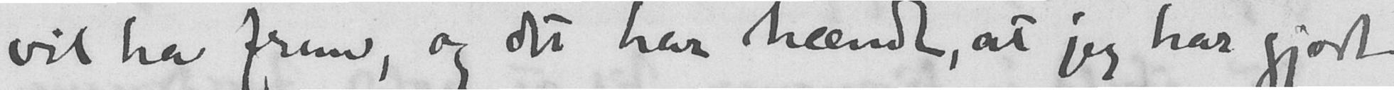vil ha frem, og det har hændt, at jeg har gjort
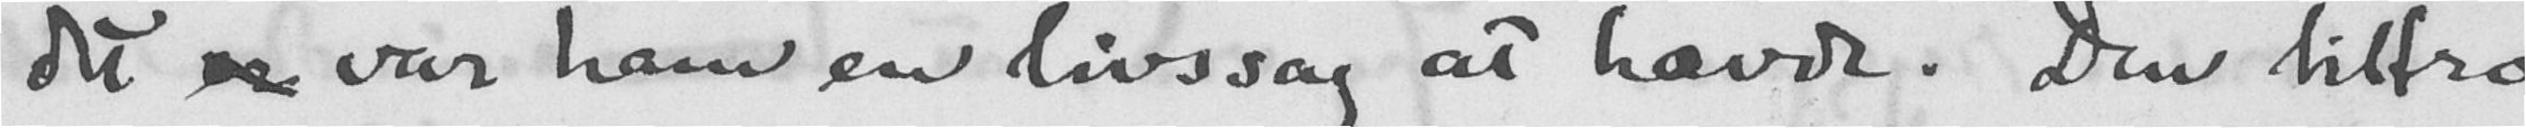det er var ham en livssag at hævde. Den tiltro
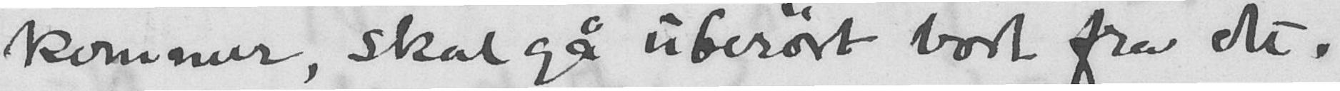kommer, skal gå uberørt bort fra det.
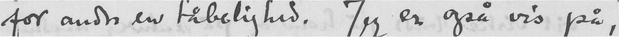for andre en tåbelighed. Jeg er også vis på,
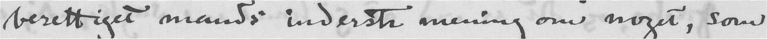berettiget mands inderste mening om noget, som
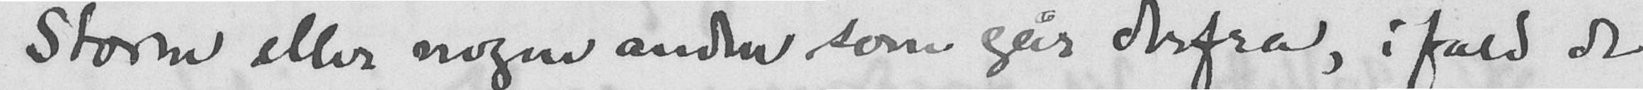Storm eller nogen anden som går derfra, ifald de
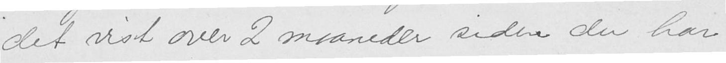det vist over 2 maaneder siden du har
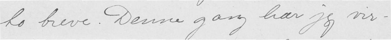to breve Denne gang har jeg vir-
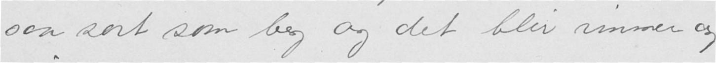saa sort som beg og det blir immer og
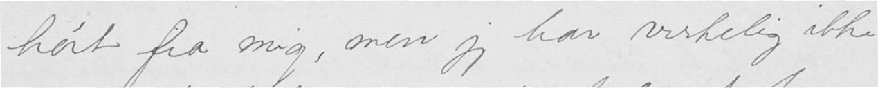hørt fra mig, men jeg har virkelig ikke
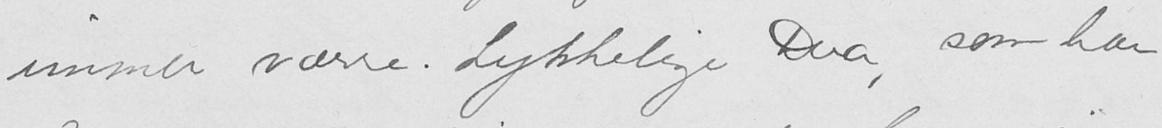immer værre. Lykkelige Dea, som har
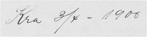Kra 3/4 - 1900
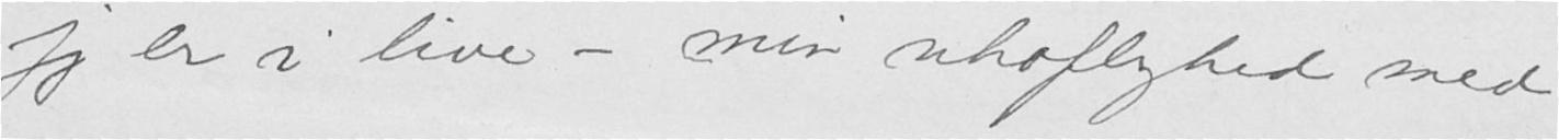jeg er i live - min uhøflighed med
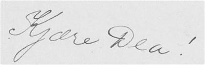Kjære Dea!
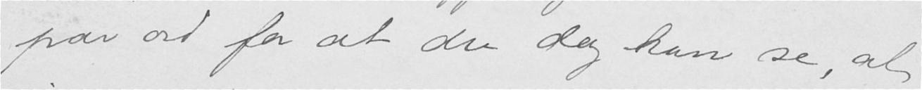par ord for at du dog kan se, at
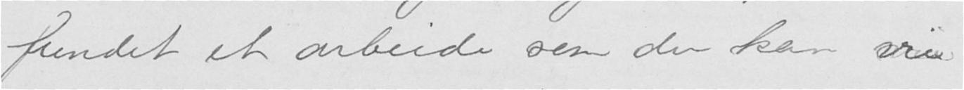fundet et arbeide som du kan vie
